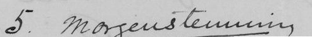5. Morgenstemning
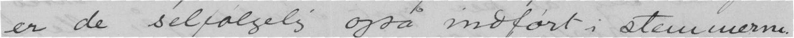er de selfølgelig også indført i stemmerne.
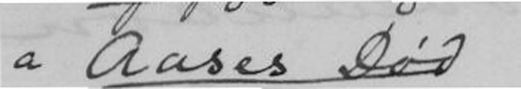a Aases Død
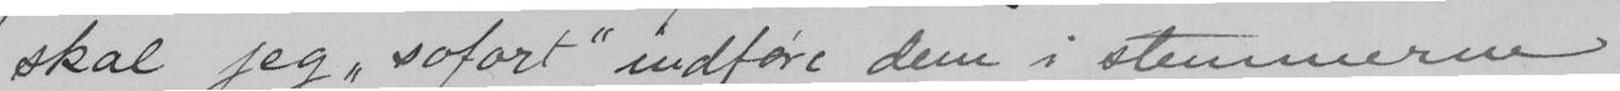skal jeg "sofort" indføre dem i stemmerne
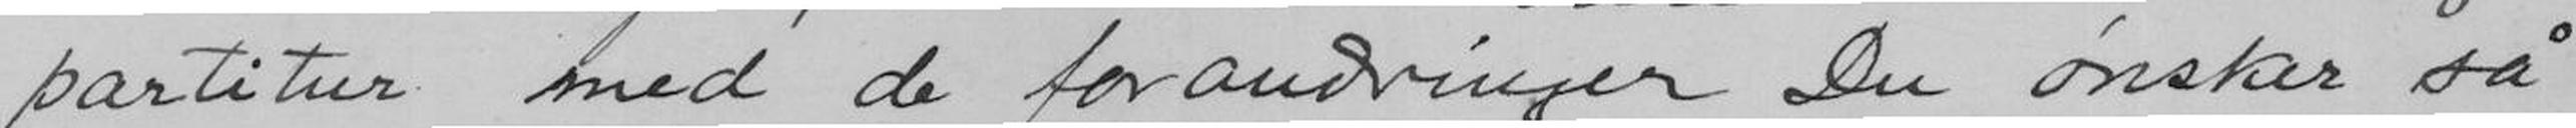partitur med de forandringer Du ønsker så
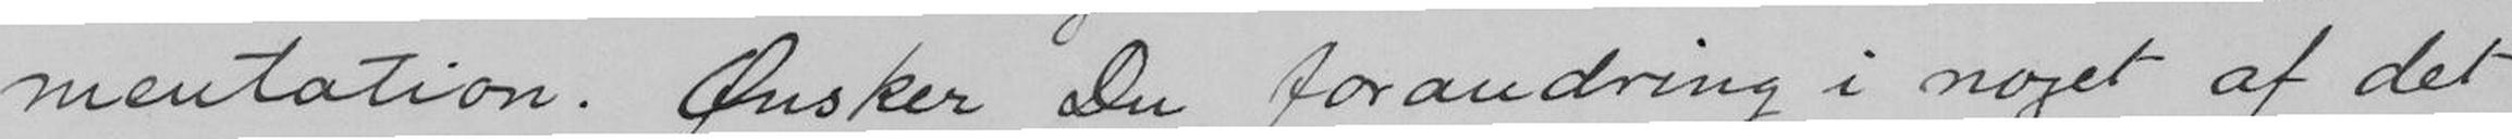mentation. Ønsker Du forandring i noget af det
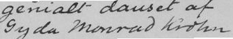Gyda Monrad Krohn
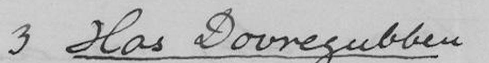3.Hos Dovregubben
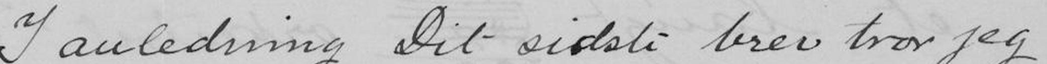I anledning Dit sidste brev tror jeg
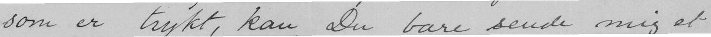som er trykt, kan Du bare sende mig et
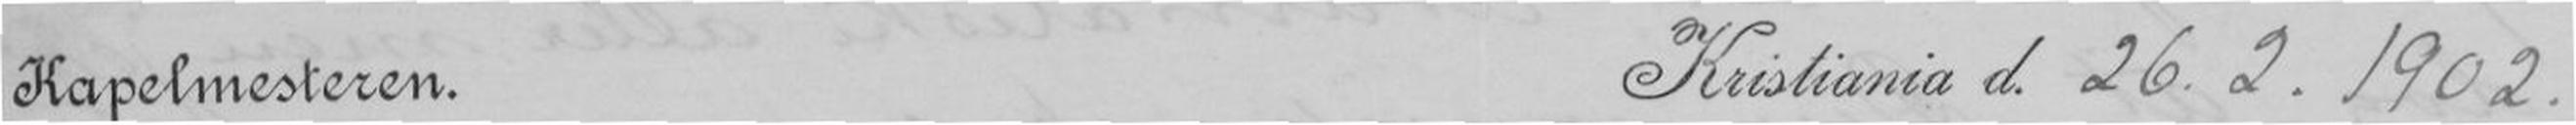Kapelmesteren. Kristiania d. 26.2.1902.
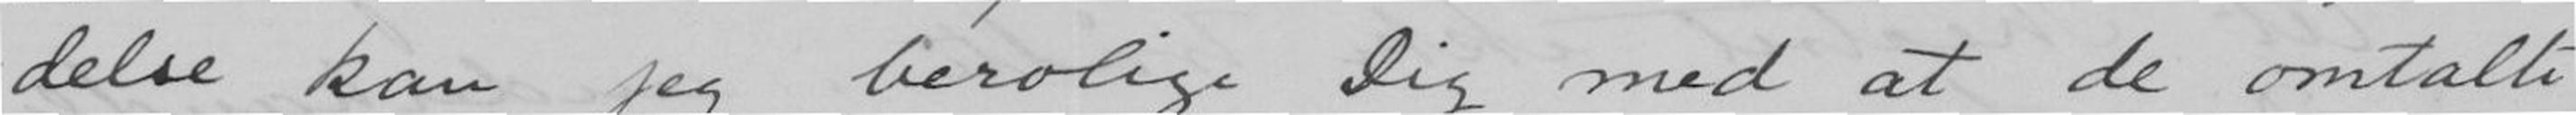delse kan jeg berolige Dig med at de omtalte
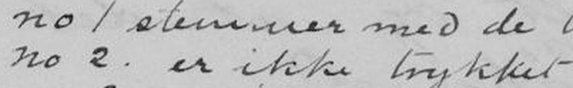no 2. er ikke trykket
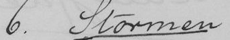6. Stormen
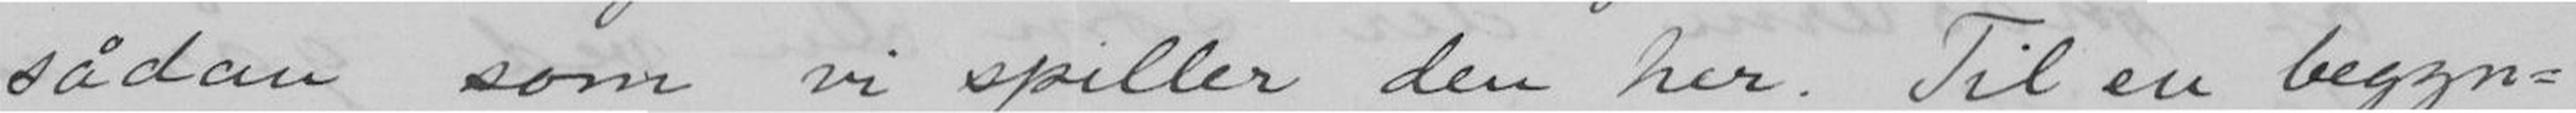sådan som vi spiller den her. Til en begyn-
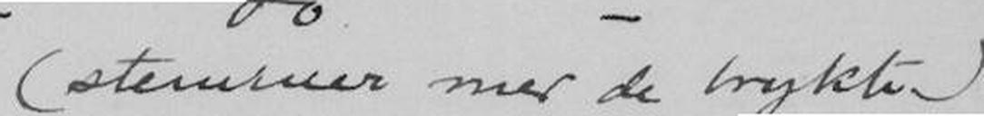(stemmer mer de trykte)
